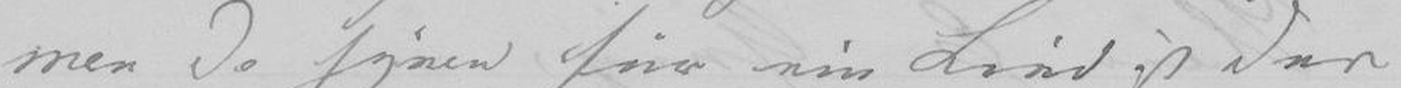men De syner fire ein Lind jo der der
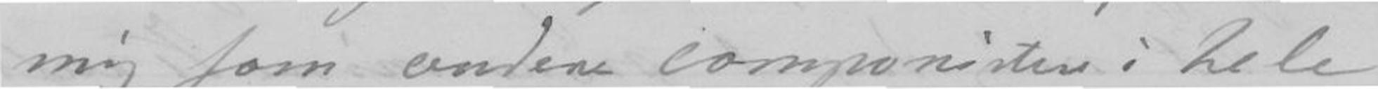mig som anden componister i hele
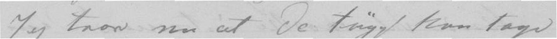Jeg tror nu at De trygt kan tage
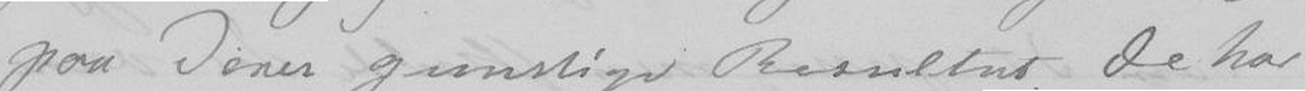paa Deres gunstige Resultat. De har
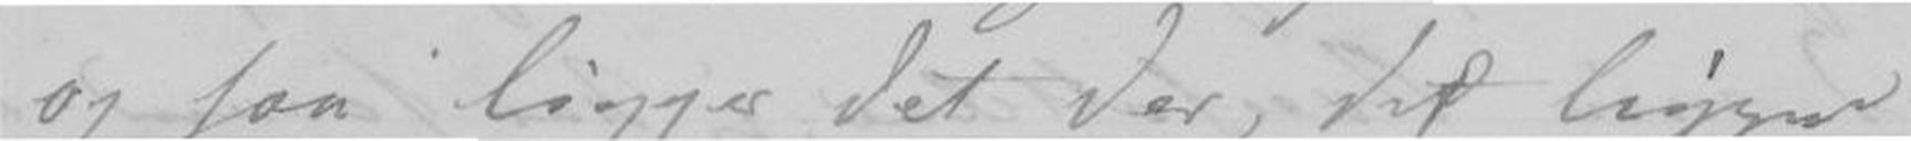og saa ligger dit der, det liggende
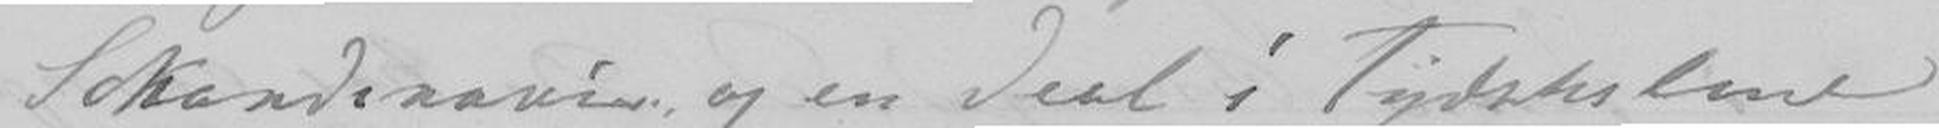Sckandinavien og en deal i Tydskslene
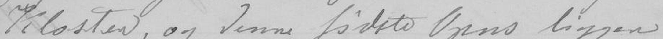Kloster, og Denne sidste Opus ligger
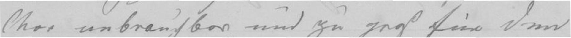Chor unbrauchbar und zu gras für dem
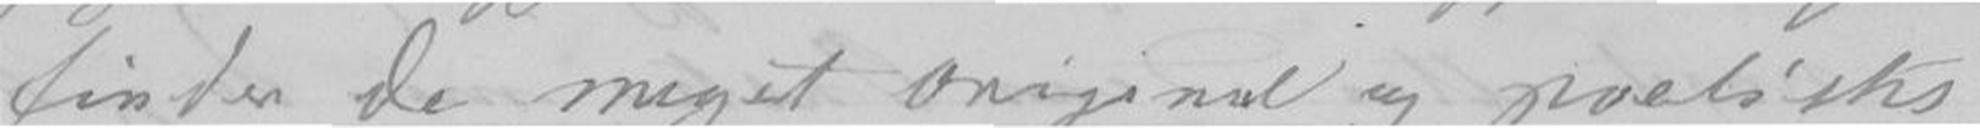finder de meget original og poetisks
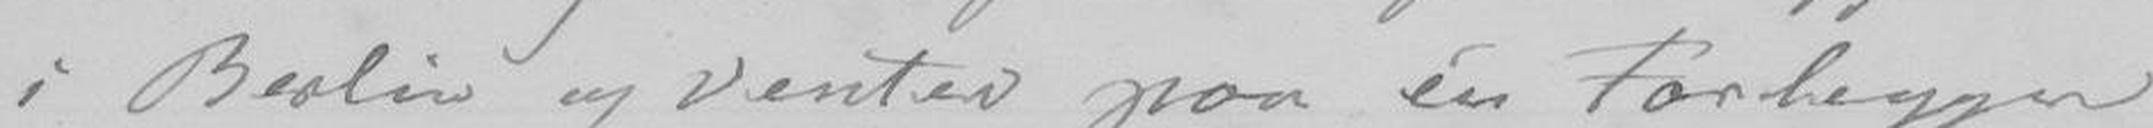i Berlin og venter paa èn Forlegger
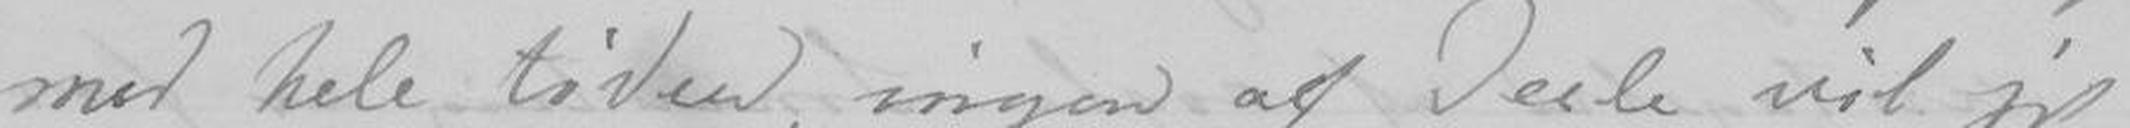med hele tiden, ingen af Dele vil jeg
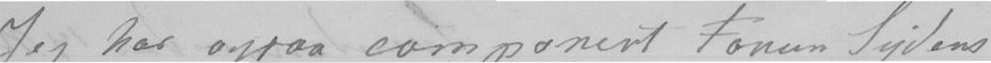Jeg har ogsaa componert Foran Sydens
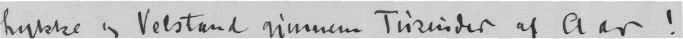Lykke og Velstand gjennem Tusender af Aar!
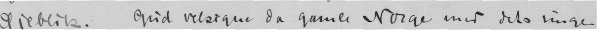Øieblik. Gud velsigne da gamle Norge med dets ringe
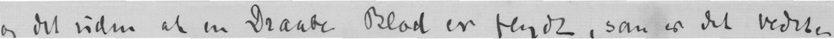og det uden at en Draabe Blod er flydt, saa er det bedste
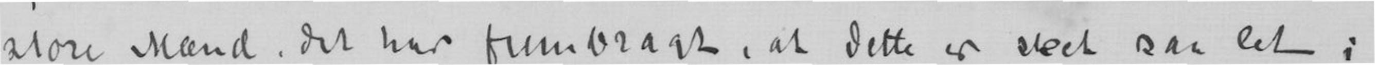store Mænd. det har frembragt, at dette er sket saa let;
